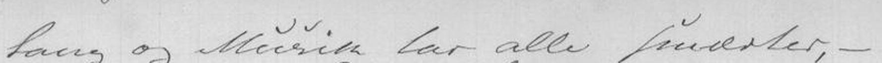Sang og Musik lar alle smærter, -
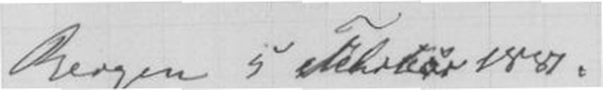Bergen 5 Februar 1881
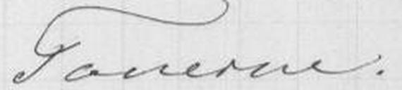Tonerne.
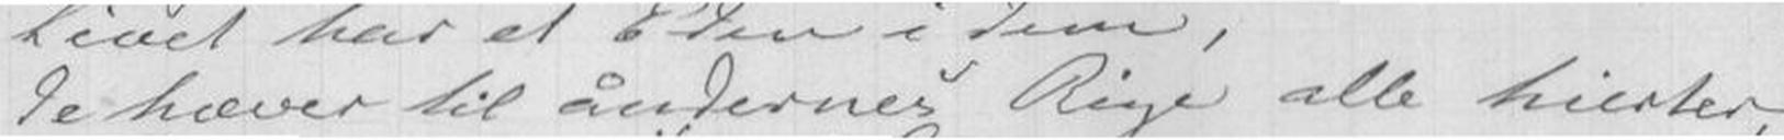De hæver til åndernes Rige alle hierter,
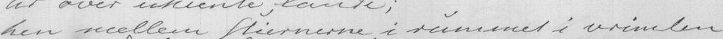hen mellem Stiernerne i rummel i vrimelen
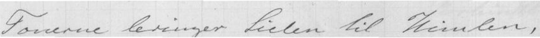Tonerne bringer siden til Himlen,
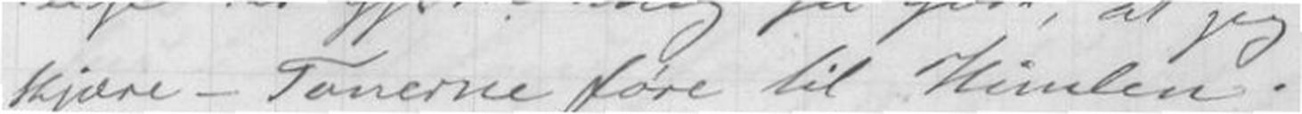kjære - Tonerne føre til Himlen.
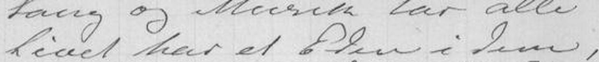livet har et Eden i Dem,
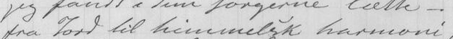fra Jord til himmelsk harmoni,
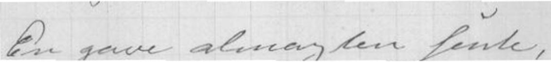En gave almagten sente
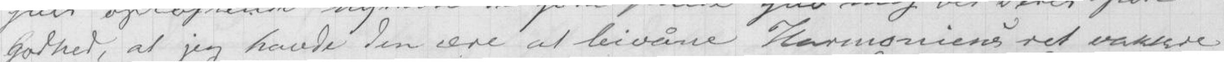Godhed, at jeg havde den ære at bivåne Harmoniens ret vakkre
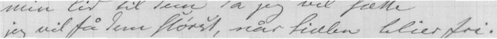jeg vil få Dem størst, når tidlen blive fri.
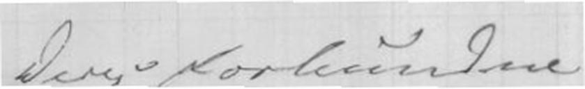Deres Forbundne
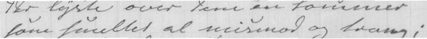som smeltet al mismod og tvang;
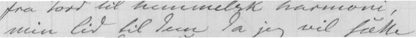min lid til Dem Da jeg vil sætte
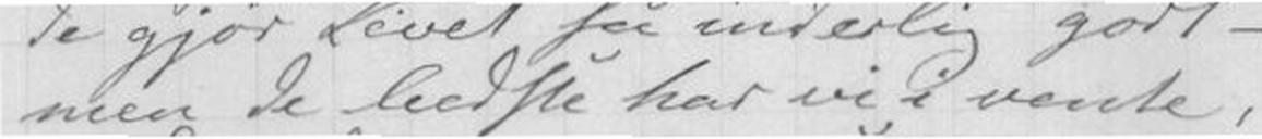men De bedste har vi i vente,
